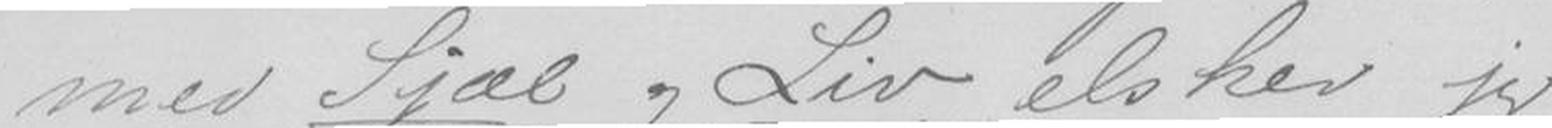med Sjæl og Liv elsker jeg
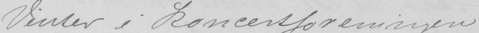vinter i koncertforeningen
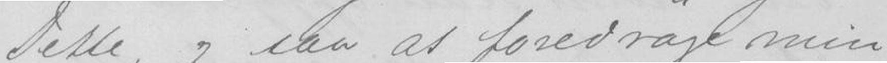Dette, og saa at foredrage min
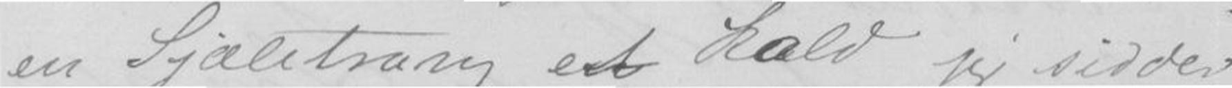en Sjæletrang, et kald jeg sidder
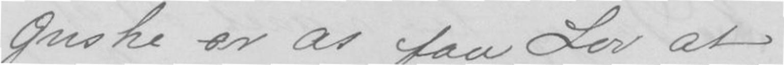Ønske er at faa Lov at
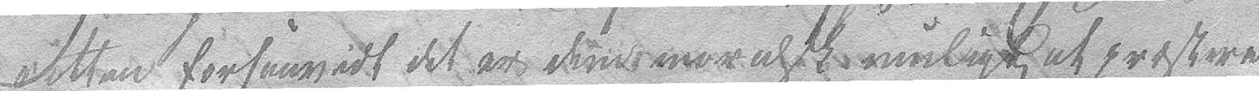retten forsaavidt det er dem moralsk muligt at præstere
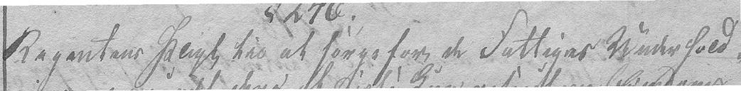Regentens Pligt til at sørge for de Fattiges Underhold-
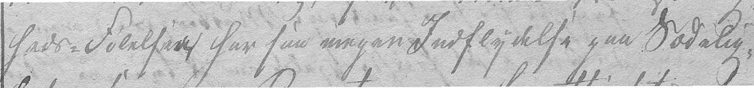heds-Følelsen har saa megen Indflydelse paa Sædelig-
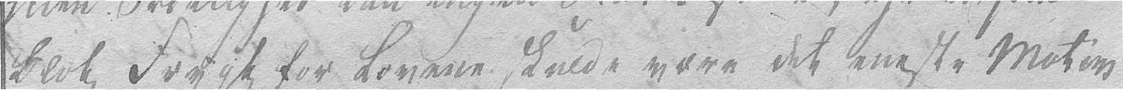blot Frygt for Lovene skulde være det eneste Motiv
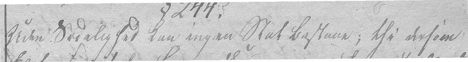Uden Sædelighed kan ingen Stat bestaae; thi dersom
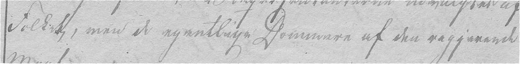Folket, men de egentlige Dommere af den regjerende
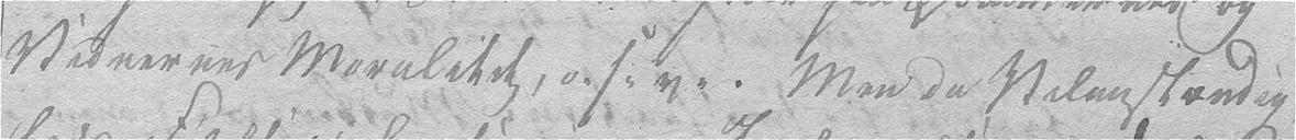Vidnernes Moralitet, o: s: v:. Men da Velanstændig-
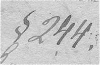§ 244.
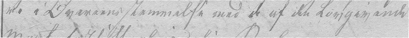re i Overeensstemmelse med de af den Lovgivende
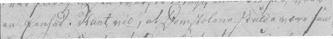en Eenhed. Kant vil, at Domstolene skulde være saa-
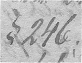§ 246.
